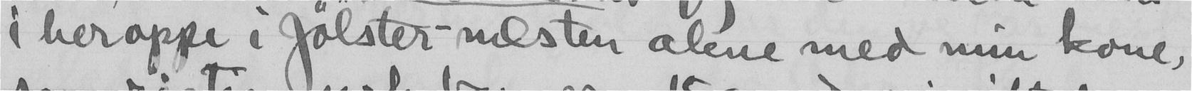i heroppe i Jølster - næsten alene med min kone,
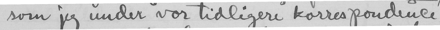som jeg under vor tidligere korrespondence
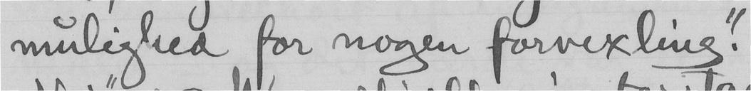mulighed for nogen forvexling."
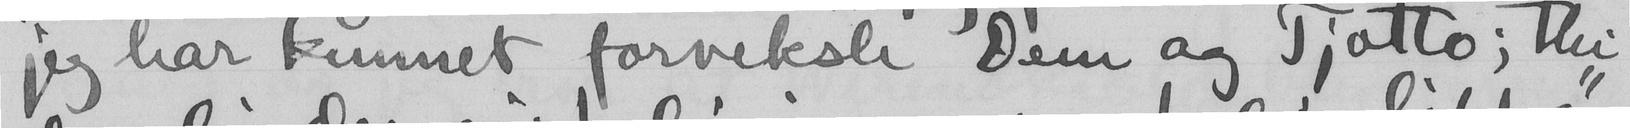jeg har kunnet forveksle Dem og Tjøttø; thi
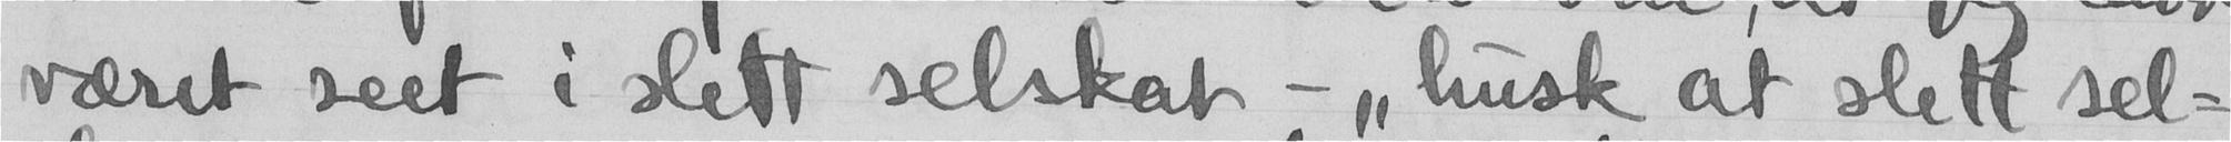været seet i slett selskab - "husk at slett sel-
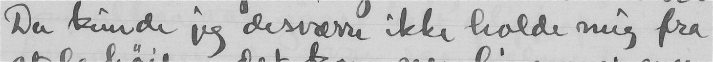Da kunde jeg desværre ikke holde mig fra
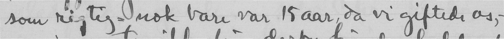som rigtig -nok bare var 15 aar, da vi giftede os,-
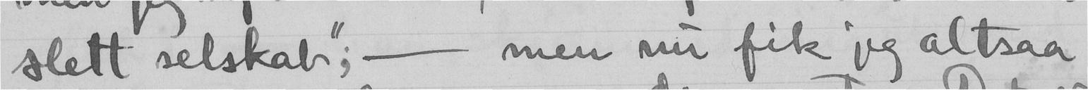slett selskab; - men nu fik jeg altsaa
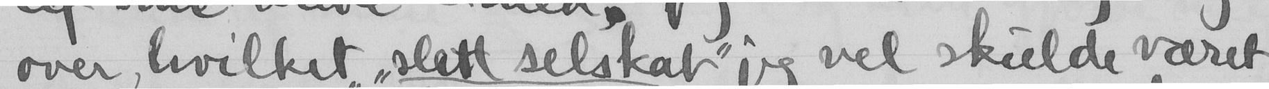over, hvilket "slett selskab" jeg vel skulde været
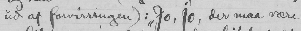ud af forvirringen): Jo, jo, der maa være
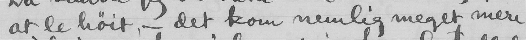at le høit, - det kom nemlig meget mere
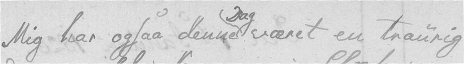Mig har ogsaa denne Dag været en traurig
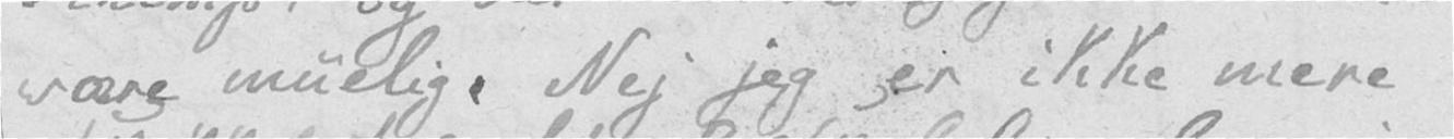være muelig. Nej jeg er ikke mere
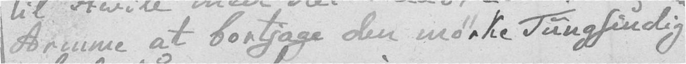Armme at bortjage den mørke Tungsindig
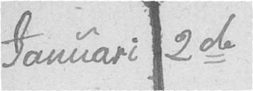Januari 2de
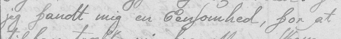jeg fandt mig en Eensomhed, for at
